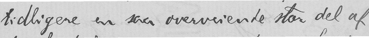tidligere en saa overveiende stor del af
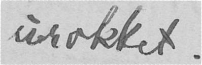urokket.
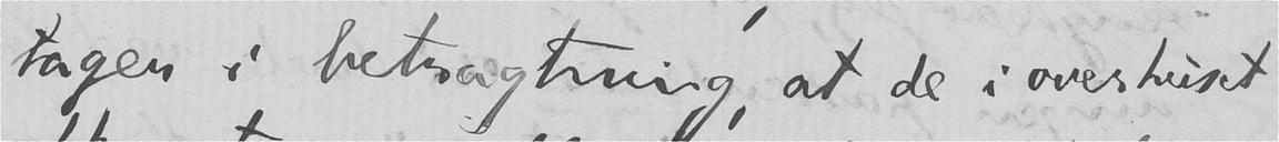tager i betragtning, at de i overhuset
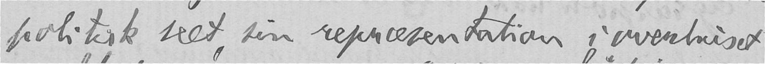politisk seet, sin repræsentation i overhuset
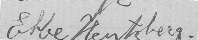Ebbe Hertzberg.
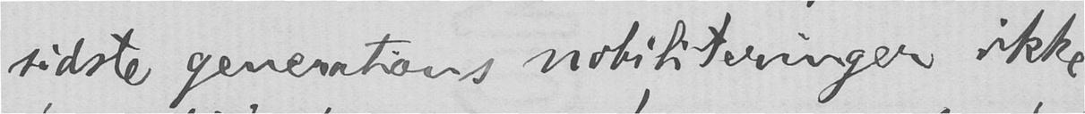sidste generations nobiliteringer ikke
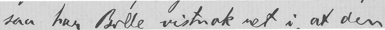saa har Bille vistnok ret i, at den
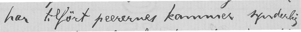har tilført peerernes kammer synderlig
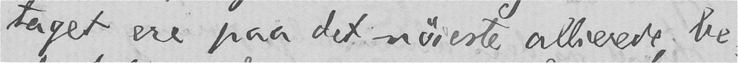taget ere paa det nøieste allierede, be-
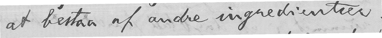at bestaa af andre ingredientser.
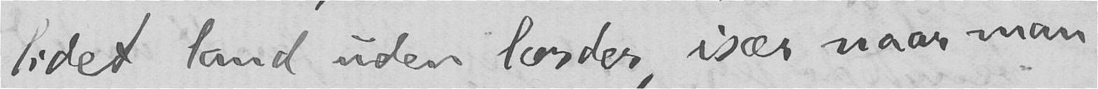lidet land uden lorder, især naar man
